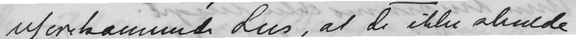uforskammede Lus, at De ikke skulde
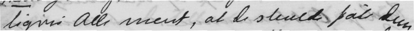ligvis Alle ment, at De skulde fåt Dem
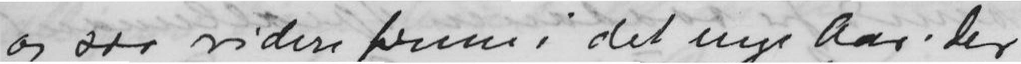og saa videre frem i det nye Aar. Der
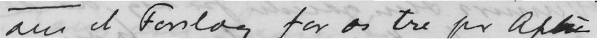om et Forslag for os tre pr Aften,
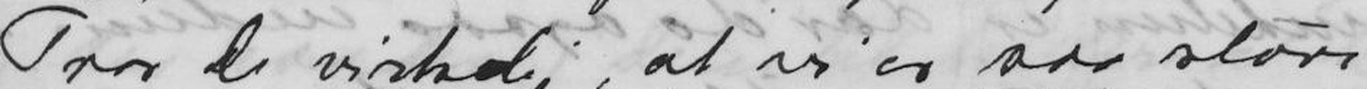Tror De virkelig, at vi er saa store
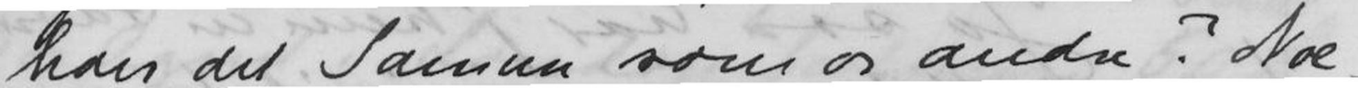have det Samme som os andre? Nae
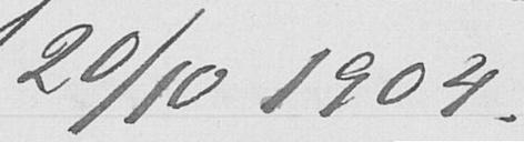20/10 1904.
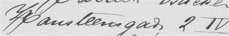Hansteensgade 2 IV
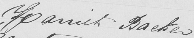Harriet Backer
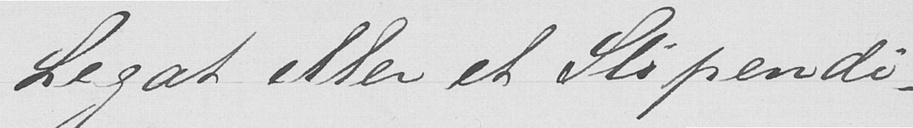Legat eller et Stipendi-
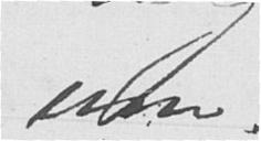um.
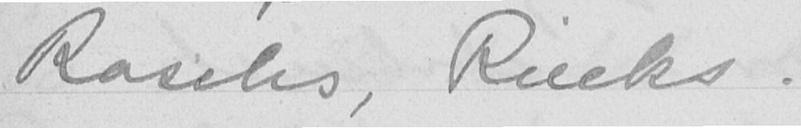Raschs, Riecks.
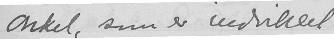onkel, som er indviklet
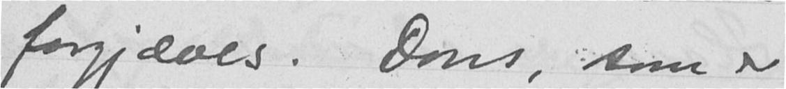forgjæves. Dons, som er
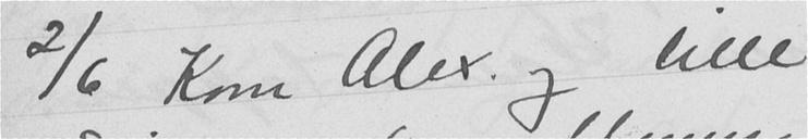2/6 Kom Alex og lille
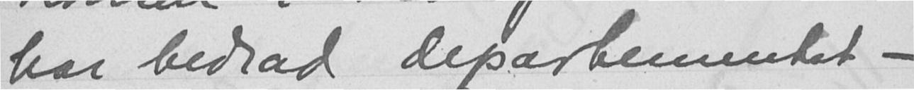har bedrad departementet -
